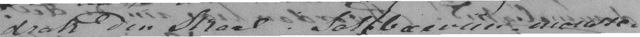drak Din Skaal i Solbærviin mousse-
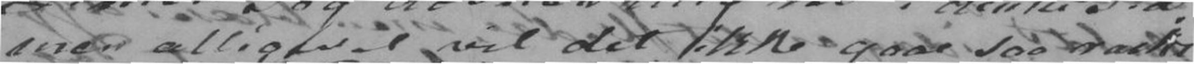men aligevel vil det ikke gae saa rask
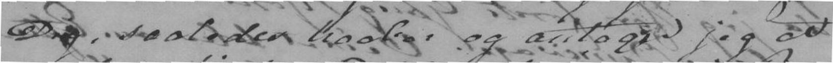Dig, saaledes haaber og antager jeg at
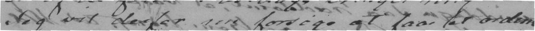Jeg vil derfor nu forsøge at faa et ordent-
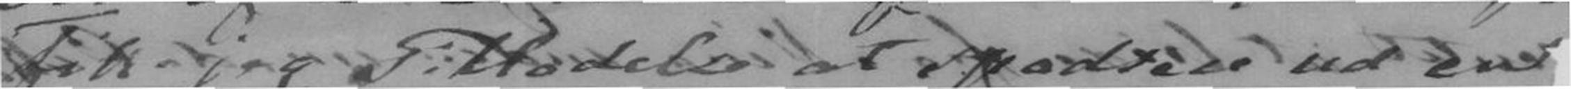fik jeg Tilladelse at spadsere ud en
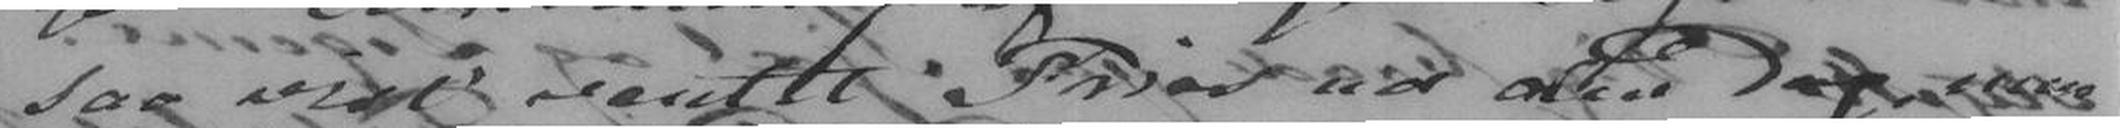saa vist ventet Fries ud den Dag, men
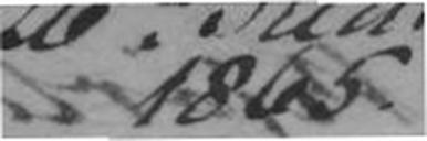1865
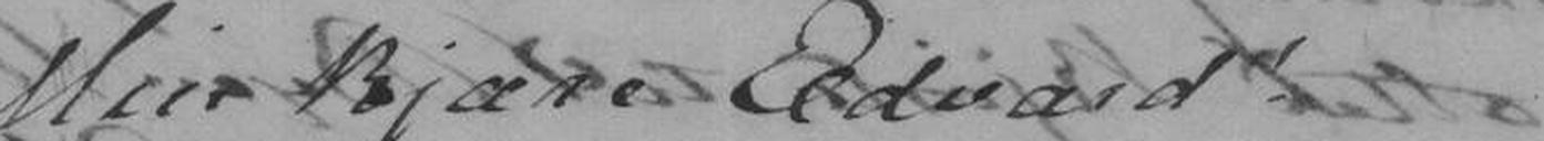Min kjære Edvard!
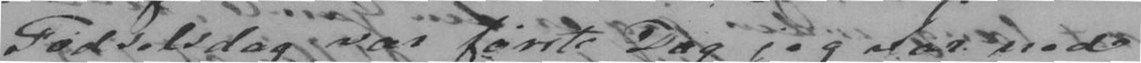Fødselsdag var første Dag jeg var nede
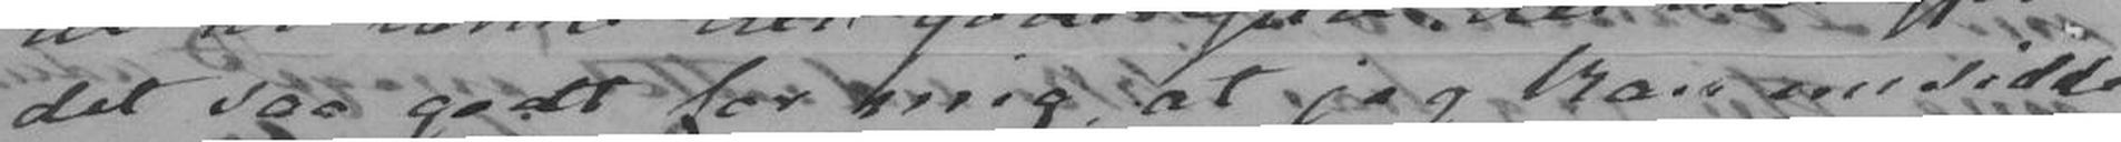det saa godt for mig, at jeg kan nu sidde
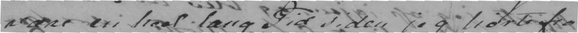være en heel lang Tid siden jeg hørte fra
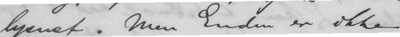lysnet. Men Enden er ikke
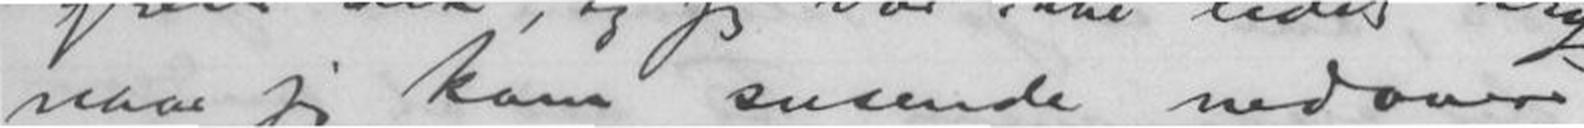naar jeg kom susende nedover
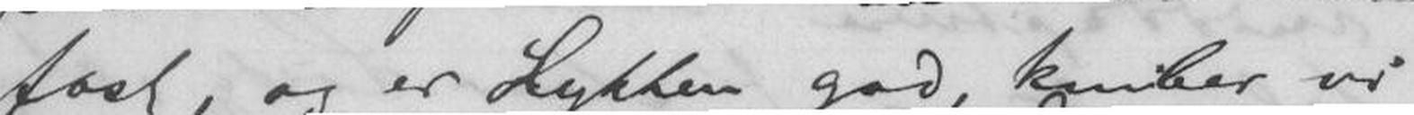fast, og er Lykken god, kanber vi
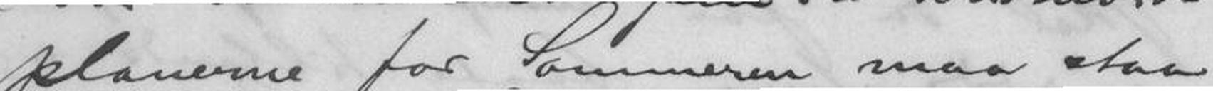planerne for Sommeren maa staa
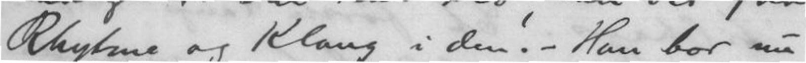Rhytme og Klang i den. - Han bor nu
